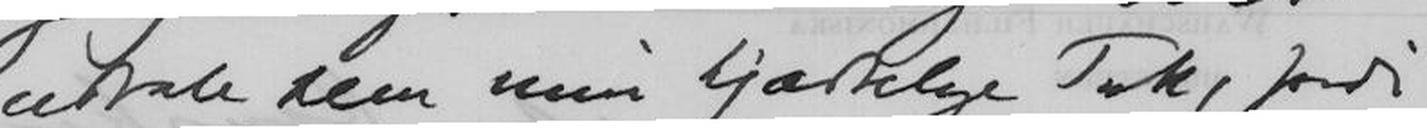udtale Dem min hjærtelige Tak, fordi
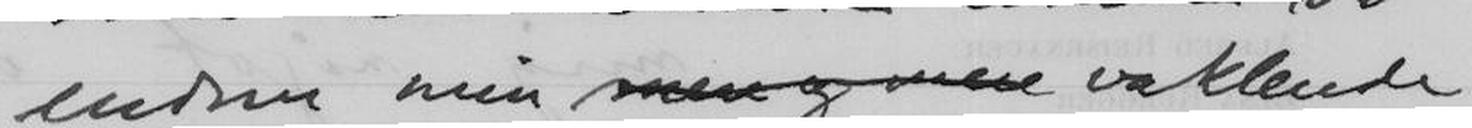endnu min mere og merevaklende
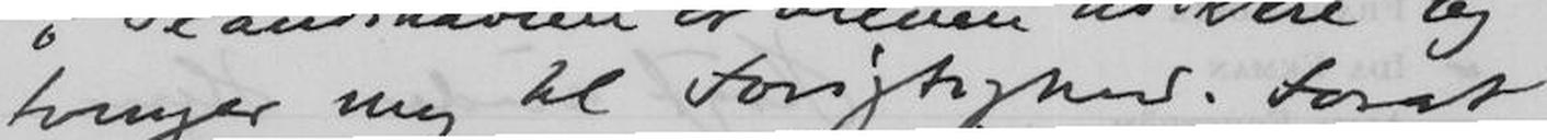tvinger mig til Forsigtighed. Forat
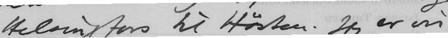Helsingfors til Høsten. Jeg er vis
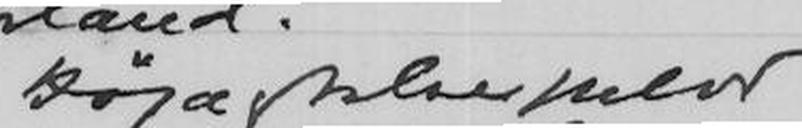Højagtelsesfuldt
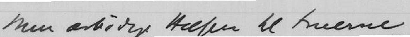Min ærbødige Hilsen til Fruerne
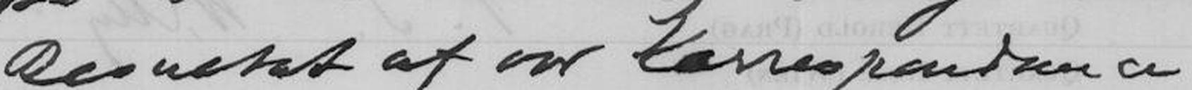Resultat af vor Korrespondance
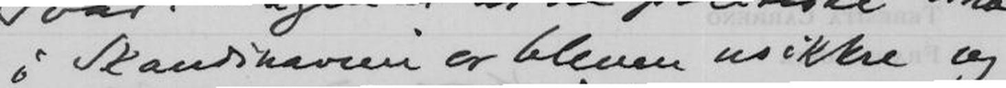i Skandinavien er bleven usikkre og
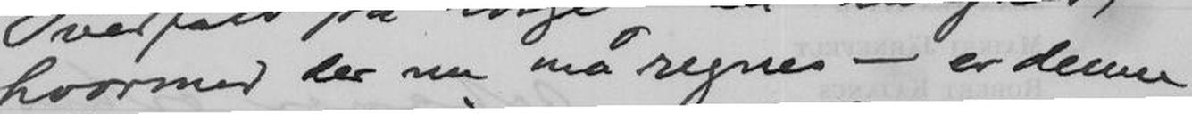hvormed der nu må regnes - er denne
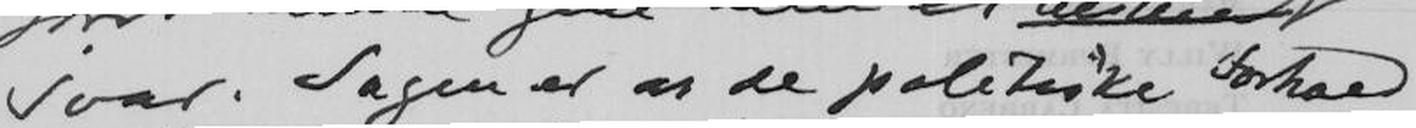Svar. Sagen er at de politiske Forhold
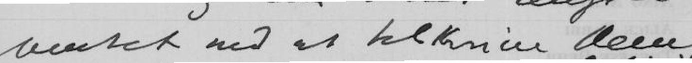ventet med at tilskrive Dem
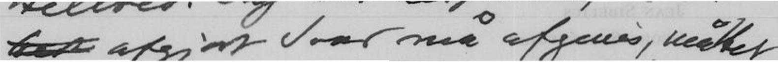afgjort Svar må afgives, måttet
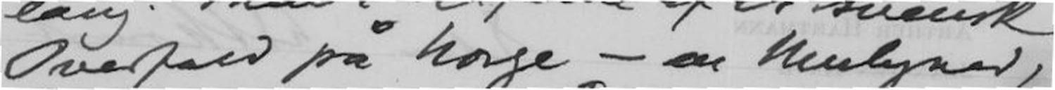Overfald på Norge - en Mulighed,
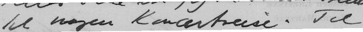til nogen Koncertreise. Til
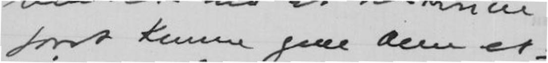forat kunne give Dem et
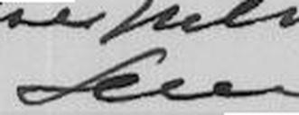Deres
